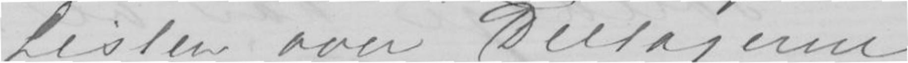Lister over Deltagerne
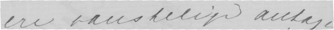ere vanskelige antager
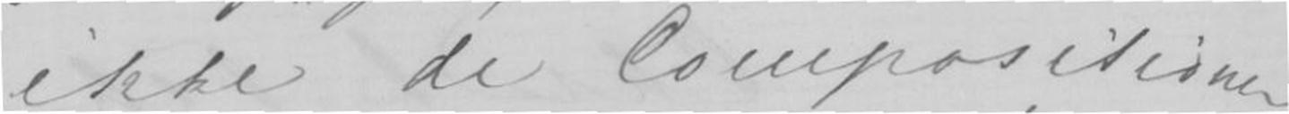ikke de Compositioner
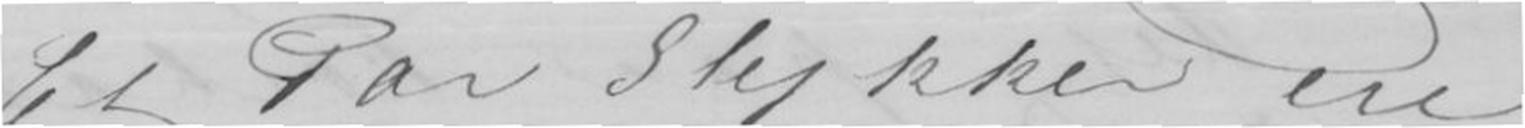Et Par Stykker ere
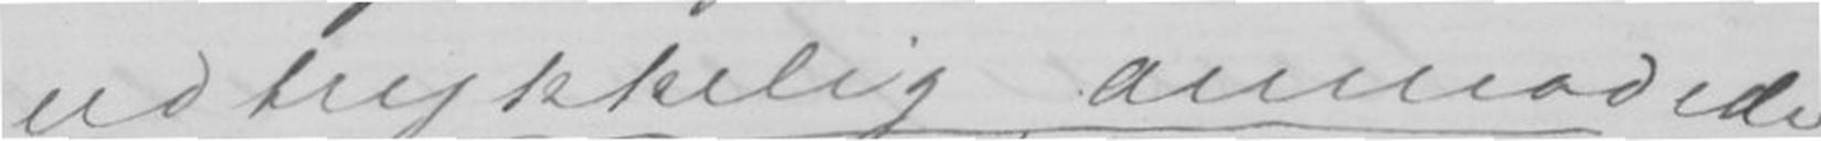udtrykkelig anmodede
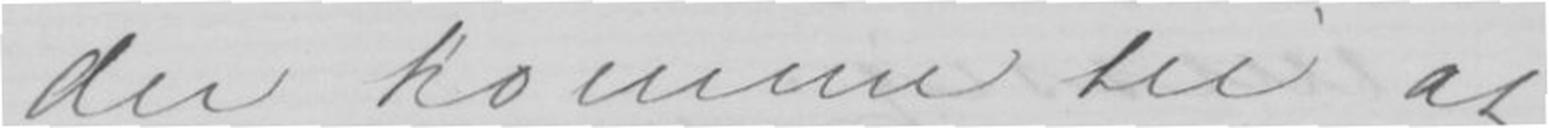der kommer til at
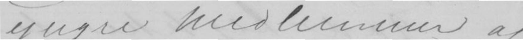yngre medlemmer af
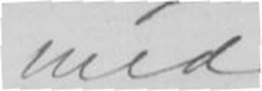med.
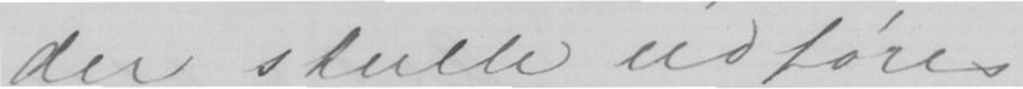der skulle udføres
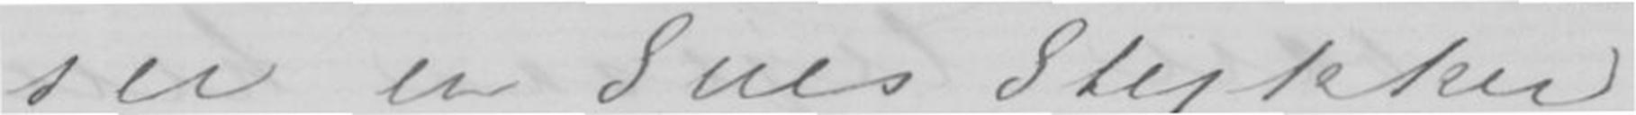ser er Snes Stykker
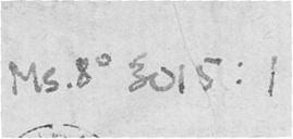Ms.8° 3015 : 1
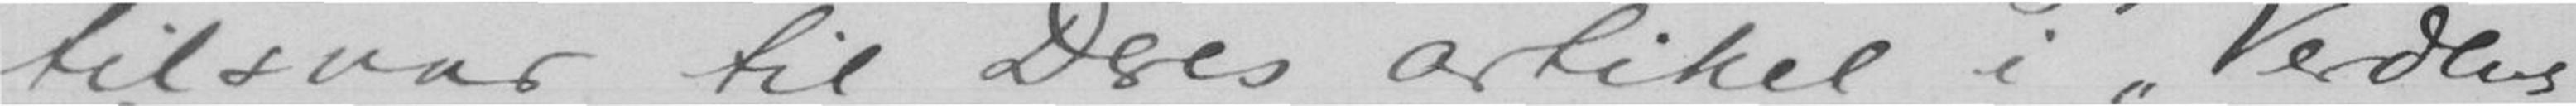tilsvar til Deres artikel i Verdens
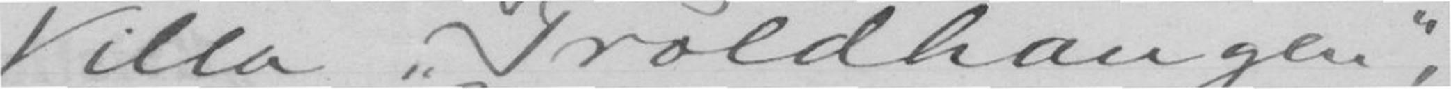Villa Troldhaugen,
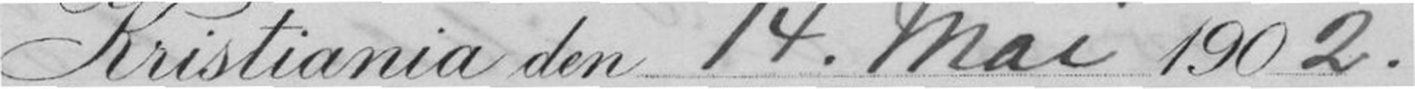Kristiania den 14. Mai 1902.
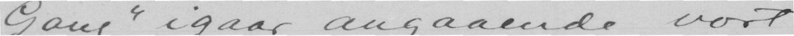Gang igaar angaaende vort
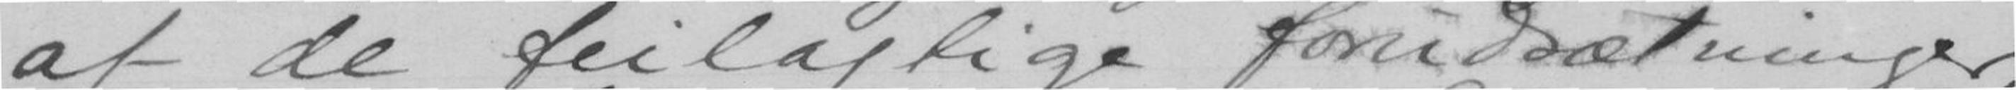af de feilagtige forudsætninger,
